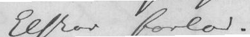Elskov forlod.
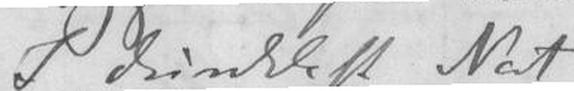I dunklest Nat
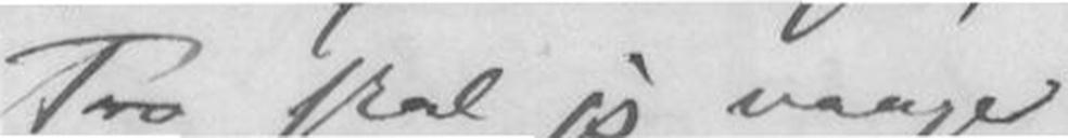Tro skal jeg vaage
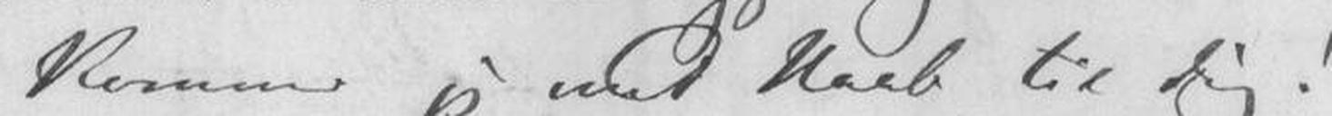Kommer jeg med Haab til dig!
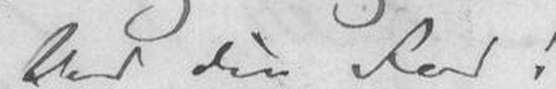Ved din Fod!
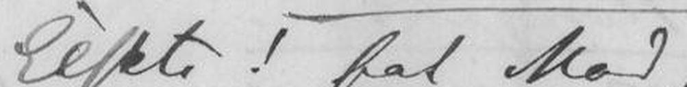Elskte! fat mod,
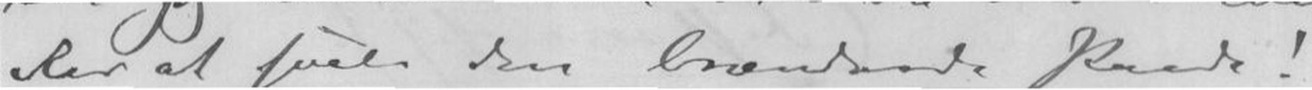For at svale den brændende Pande!
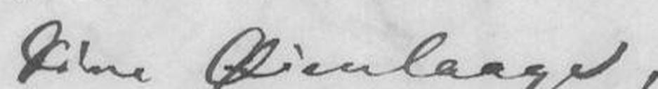Dine Øienlaage,
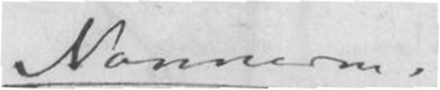Nonnerne.
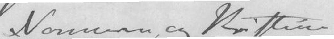Nonnerne og Kristina.
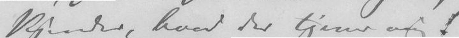Kjender, hvad der tjener mig!
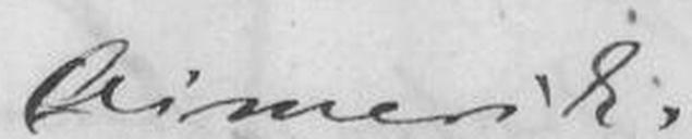Aimerik.
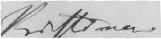Kristina.
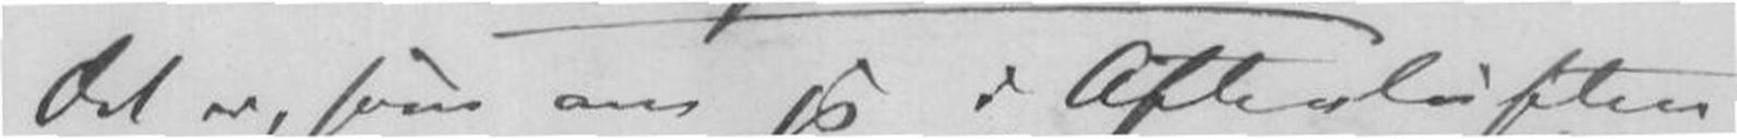Det er som om jeg i Aftenluften
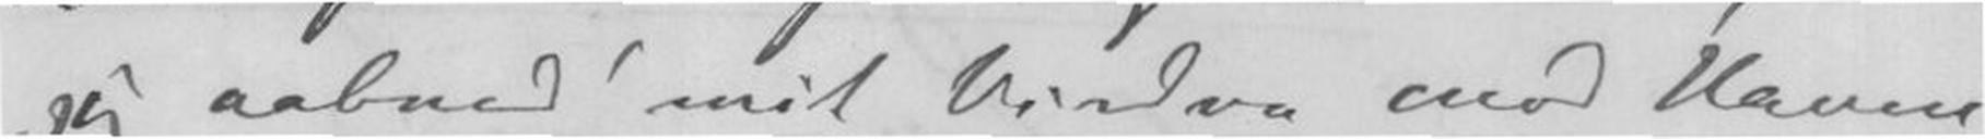jeg aabned mit Vindou mod Havne
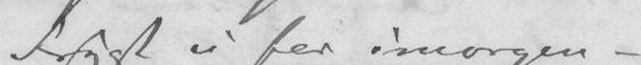Frygt ei for imorgen -
